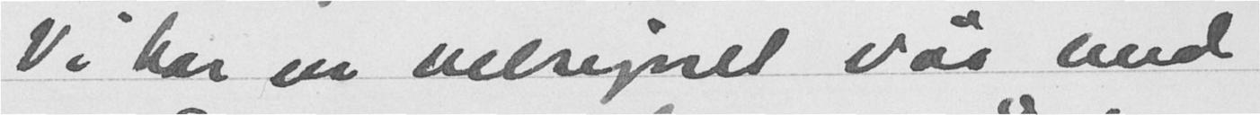Vi har en velsignet vår med
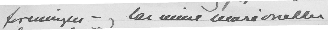foreningen - og lar mine marionetter
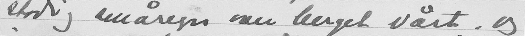stadig småregn over berget vårt. Og
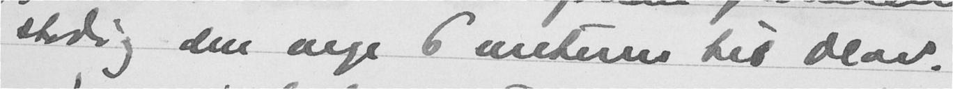stadig den nye 6 meteren til Olav.
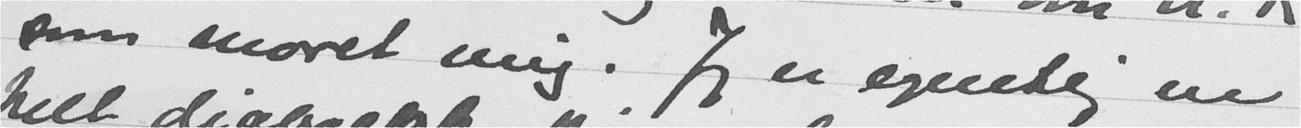som moret mig. Jeg er egentlig en
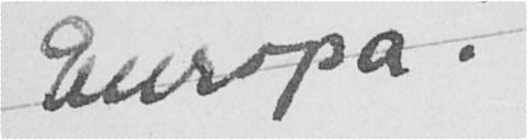Europa!
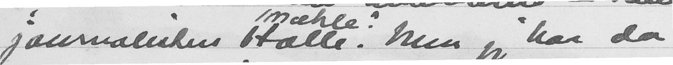journalisten Halle/Mæhle? Men jeg har da
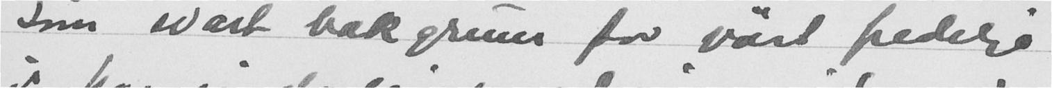Som svart bakgrunn for vårt fredelige
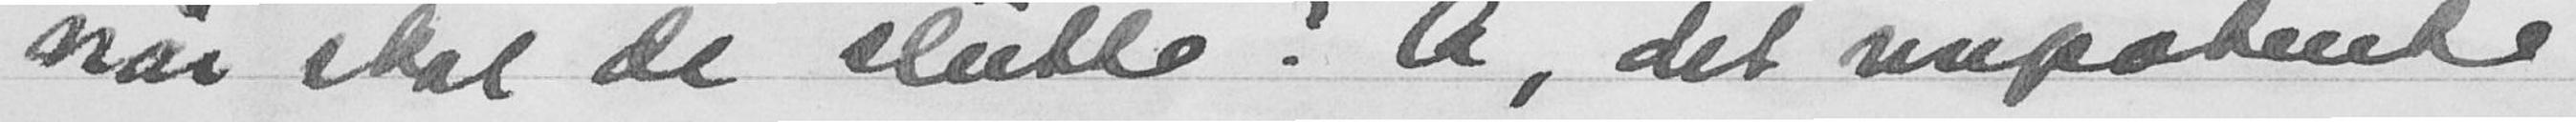når skal de slutte? Å, det impotente
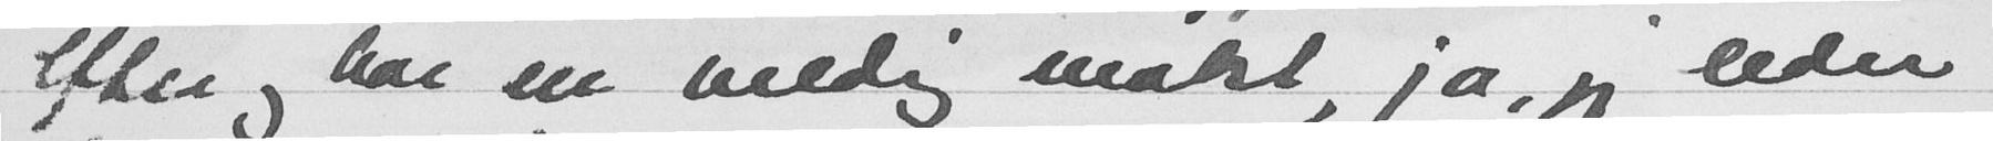efter og har en veldig makt, ja, jeg leder
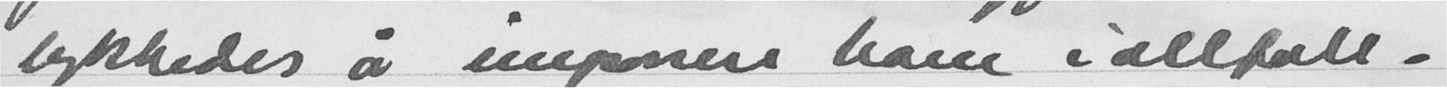lykkedes å imponere ham i allfall.
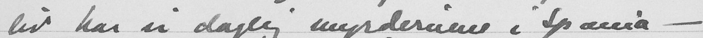liv har vi daglig myrderiene i Spania -
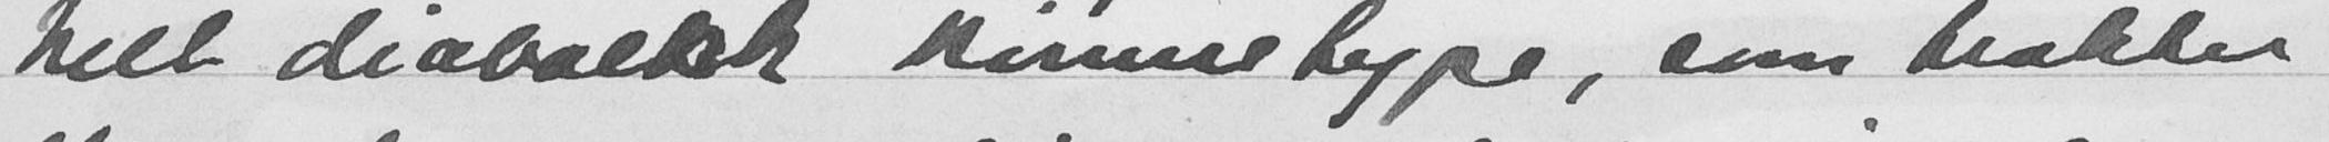helt diabolsk kvinnetype, som trakter
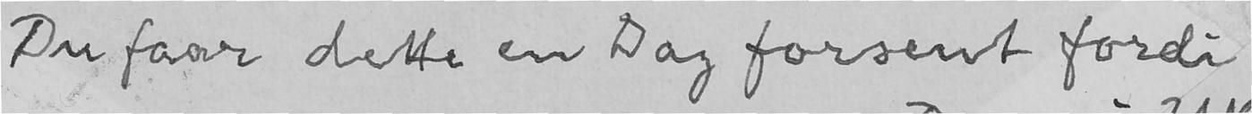Du faar dette en Dag forsent fordi
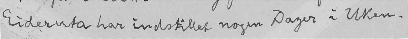Eideruta har indstillet nogen Dager i Uken.
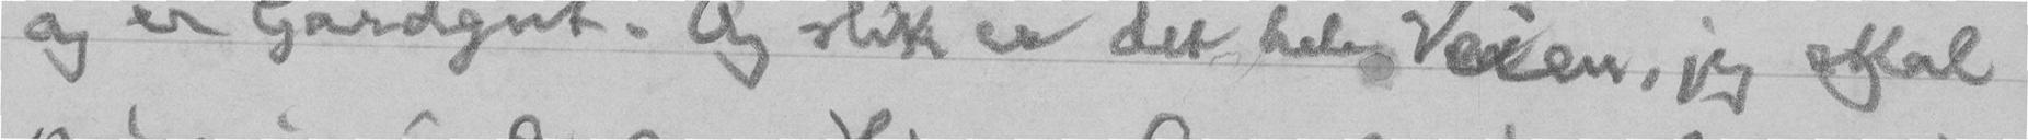og er Gardgut. Og slik er det hele Veien, jeg skal
